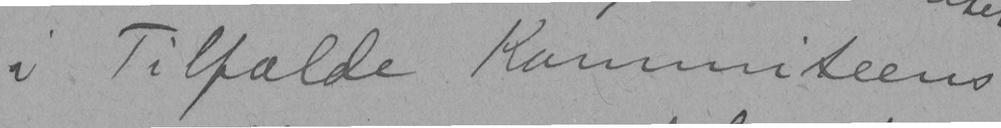i Tilfælde Kommiteens
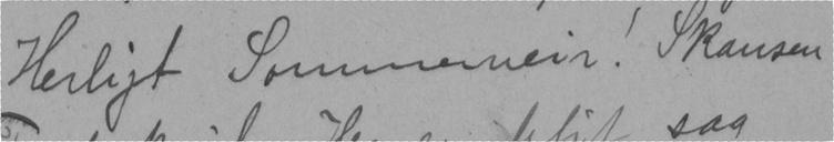Herligt Sommerveir! Skansen
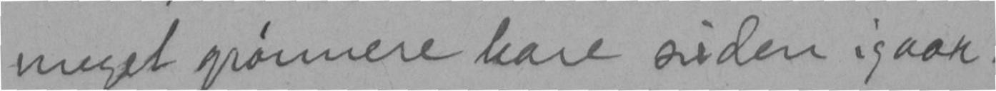meget grønnere bare siden igaar.
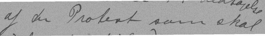af en Protest som skal
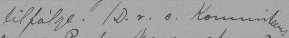tilfølge. (D. v. s. Kommiteens
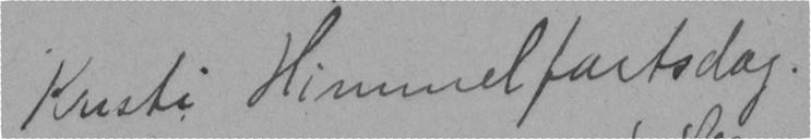Kristi Himmelfartsdag.
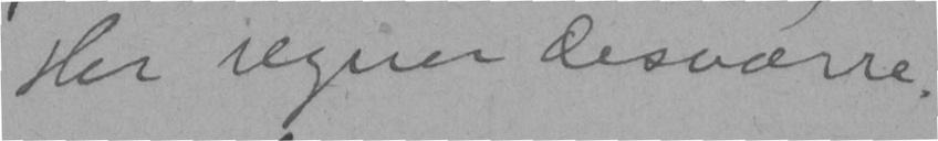Her regner desværre.
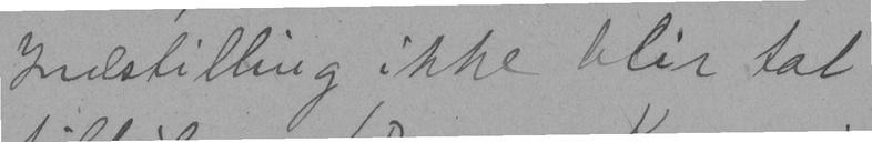Indstilling ikke blir tat
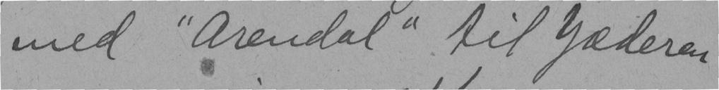med "Arendal" til Jæderen
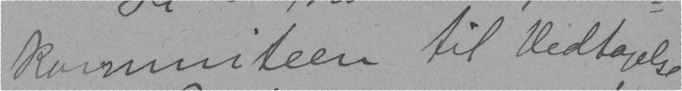kommiteen til vedtagelse
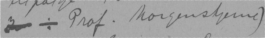Prof. Morgenstjerne)
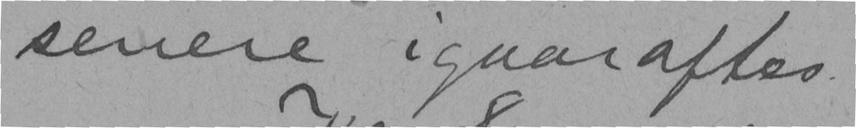senere igaaraftes.
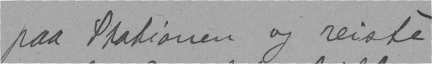paa Stationen og reiste
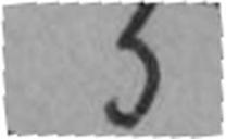5
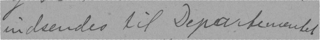indsendes til Departementet
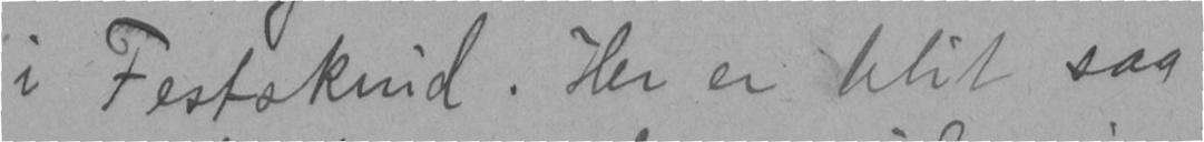i Festskrud. Her er blit saa
